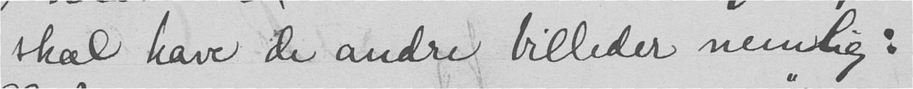skal have de andre billeder nemlig:
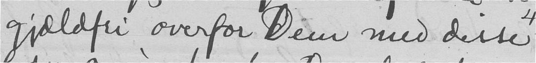gjældfri overfor Dem med disse
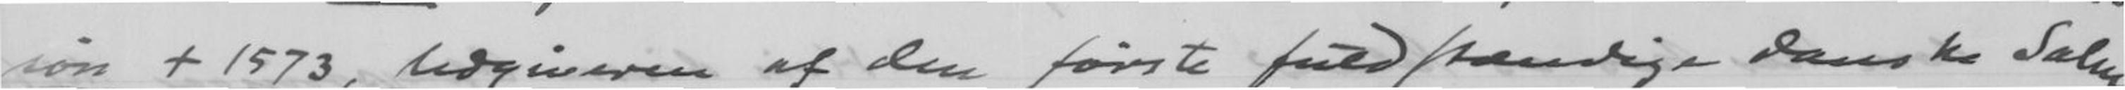søn + 1573, Udgiveren af den første fuldstændige danske Salme-
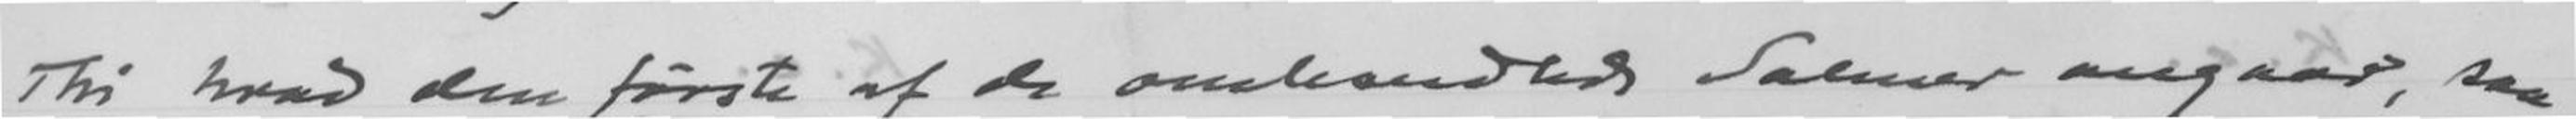Thi hvad den første af de omhandlede Salmer angaar, saa
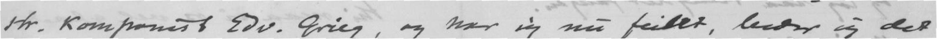Hr. Komponist Edv. Grieg, og har jeg nu feilet, beder jeg det
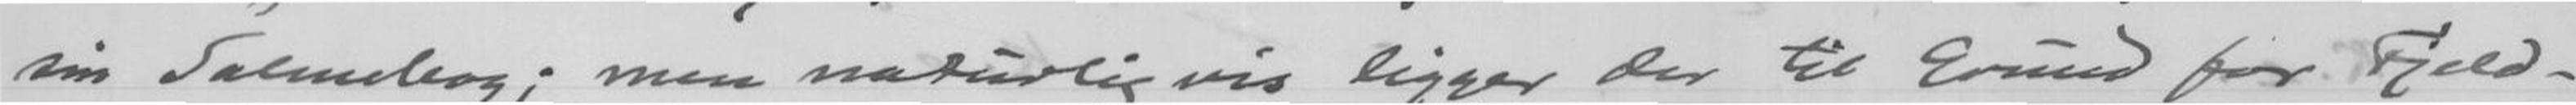sin Salmebog; men naturligvis ligger der til Grund for Fjeld-
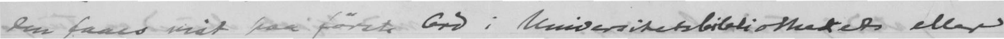den faaes vist paa første Ord i Univærsitetsbibliotekets eller
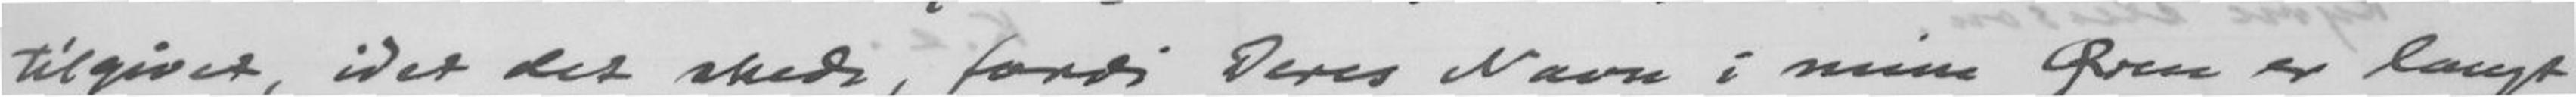tilgivet, idet det skede, fordi Deres Navn i mine Øren er langt
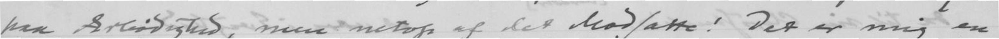paa Ærbødighed, men netop af det Modsatte! Det er mig en
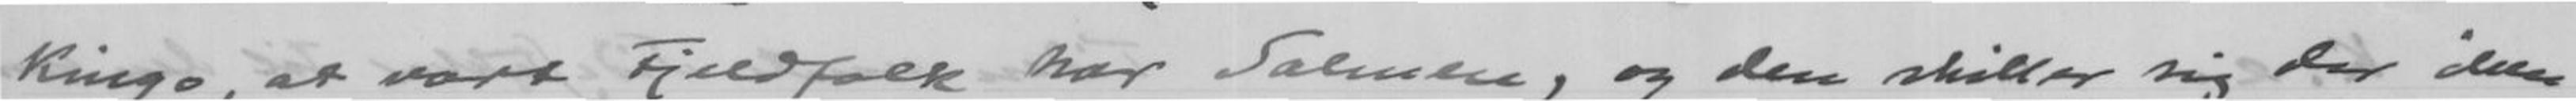Kingo, at vort Fjeldfolk har Salmen, og den skiller sig ikke
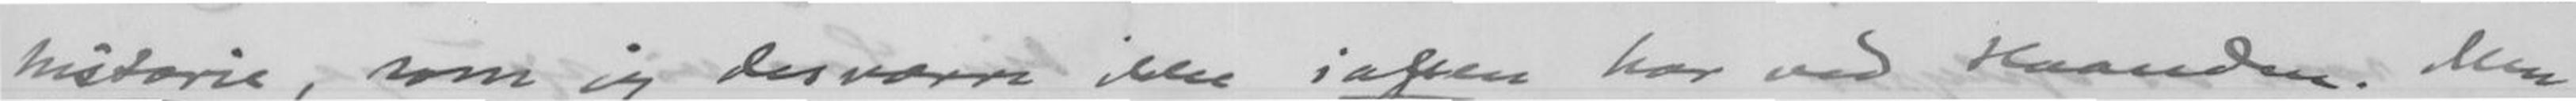historie, som jeg desværre ikke iaften har ved Haanden. Men
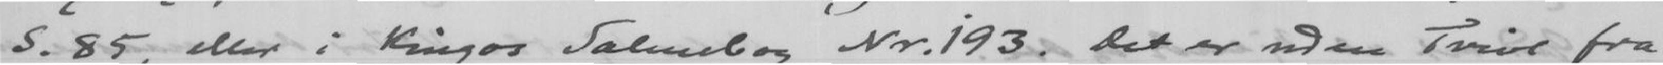S. 85, eller i Kingos Salmebog Nr. 193. Det er uden Tvivl fra
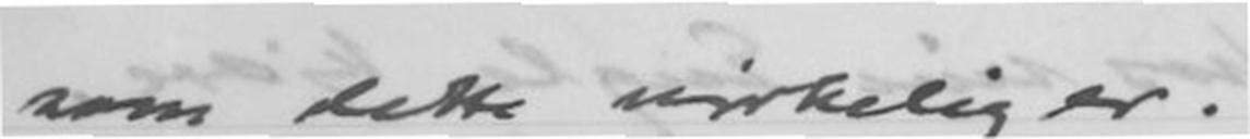som dette virkelig er.
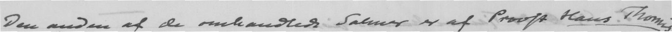Den anden af de omhandlede Salmer er af Proost Hans Thomis-
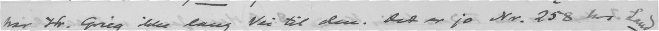har Hr. Grieg ikke lang Vei til den. Det er jo Nr. 258 hos Land-
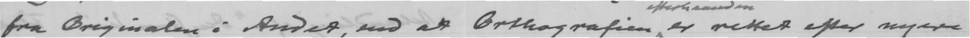fra Originalen i Andet, end at Orthografien er rettet efter nyere
